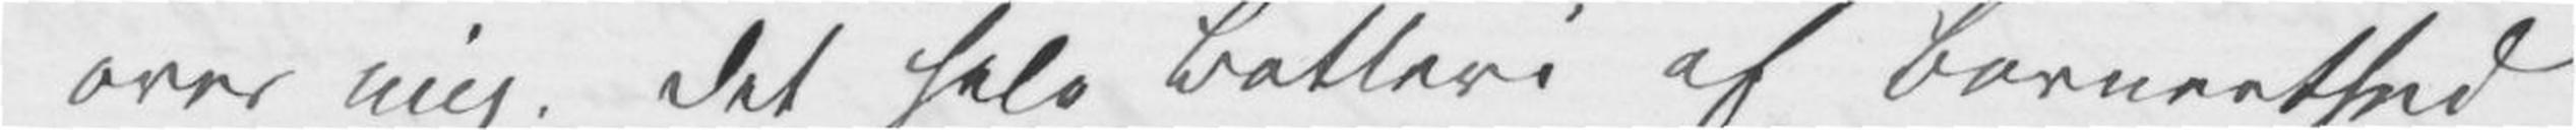over mig. Det hele Batteri af Bornerthed
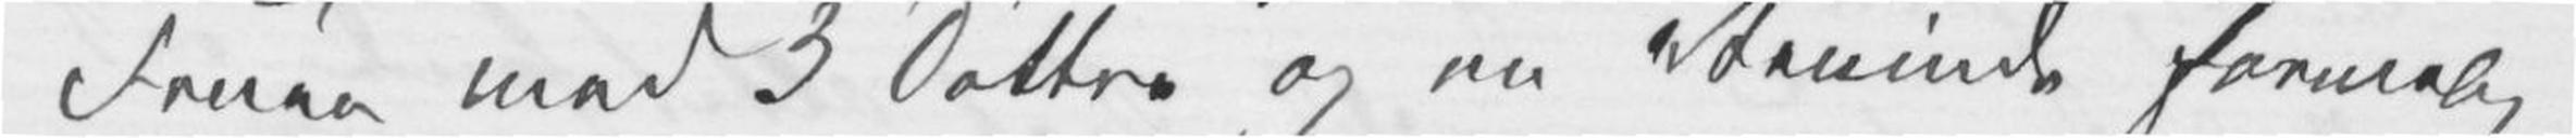Fruen med 3 Døttre og en Veninde formelig
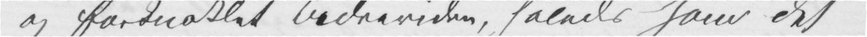og forkvaklet Bedreviden, således som det
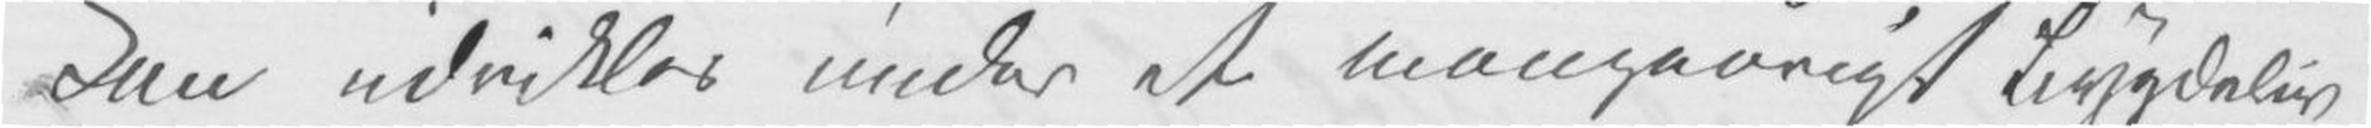kan udvikles under et mangeårigt Bygdeliv
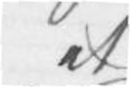et
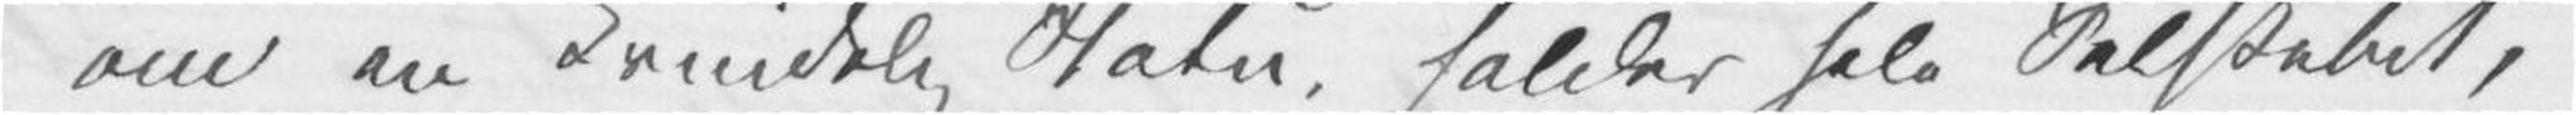om en kvindelig Statu, falder hele Selskabet,
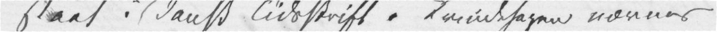stået i dansk Tidsskrift. Kvindesagen nævnes
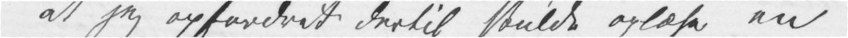at jeg opfordret dertil skulde oplæse en
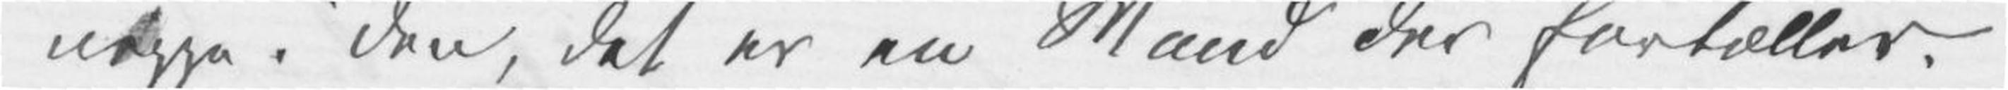neppe i den, det er en Mand der fortæller.
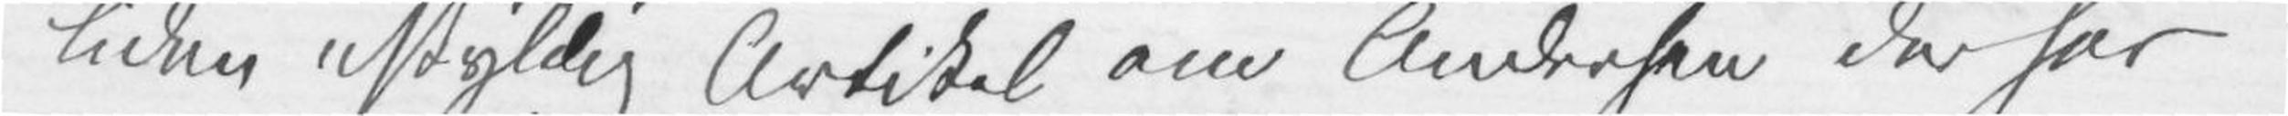liden uskyldig Artikel om Andersen der har
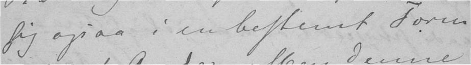sig ogsaa i en bestemt Form
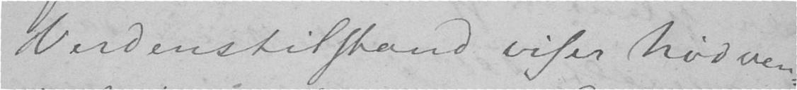Verdenstilstand viser Nødven-
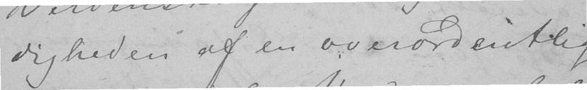digheden af en overordentlig
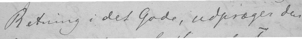Retning i det Gode, udpræger den
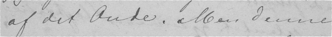af det Onde. Men denne
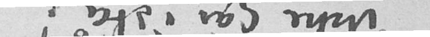ikke går istå!
